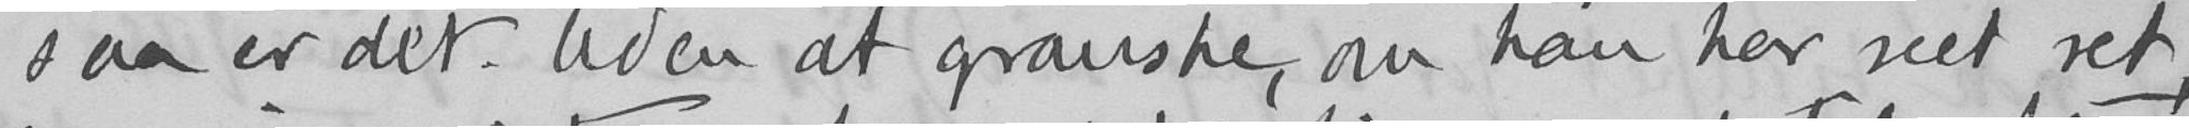saa er det. Uden at granske, om han har seet ret,
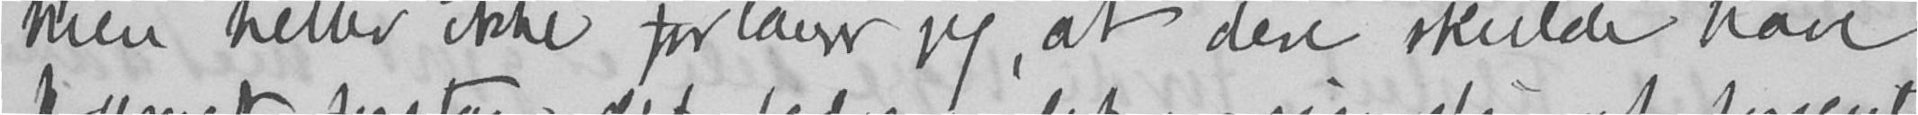Men heller ikke forlanger jeg, at dere skulde have
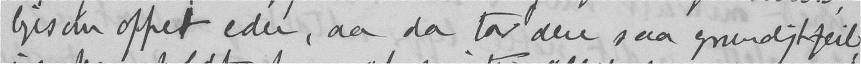ligesom oppet eder, aa da tar dere saa grundigt feil
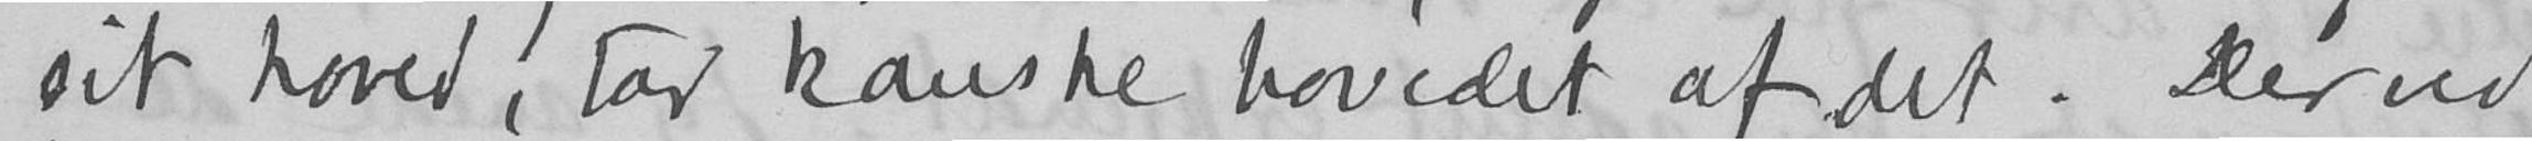sit hoved, tar kanske hovedet af det. Derved
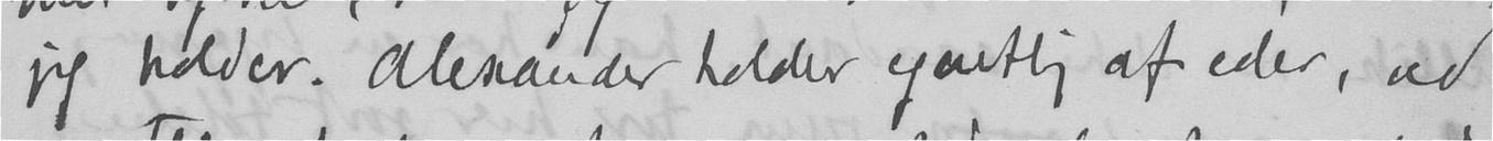jeg holder. Alexander holder egentlig af eder, ved
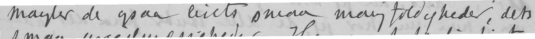mangler de ogsaa livets smaa mangfoldigheder, dets
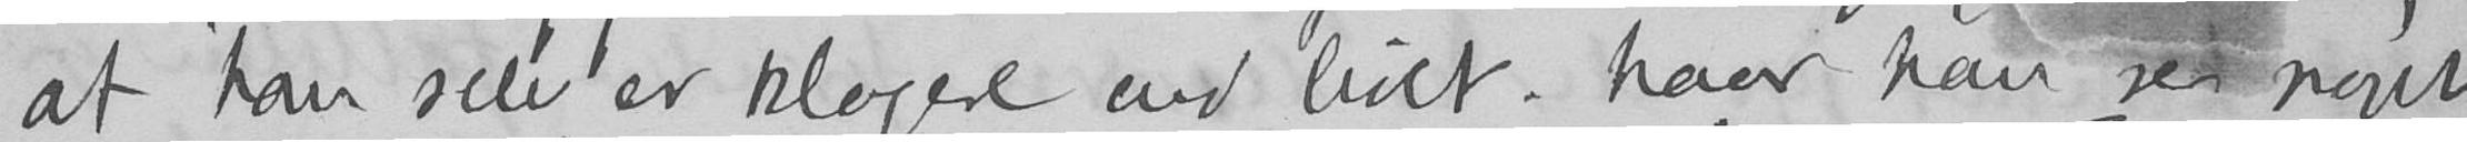at han selv er klogere end livet. Naar han ser noget,
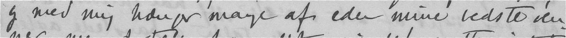og med mig hænger mange af eder mine bedste ven-
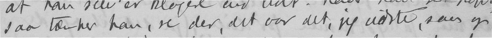saa tænker han, se der, det var det, jeg vidste, saa og
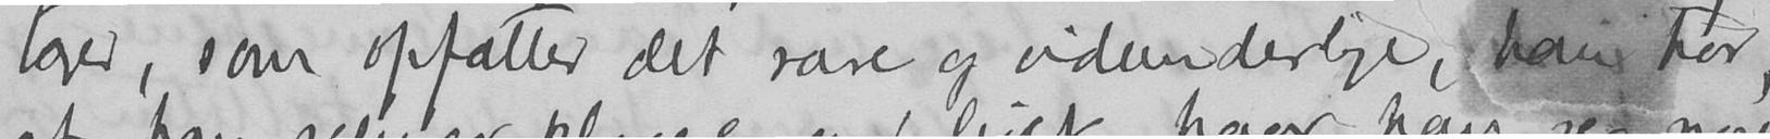tager, som opfatter det rare og vidunderlige, han tror,
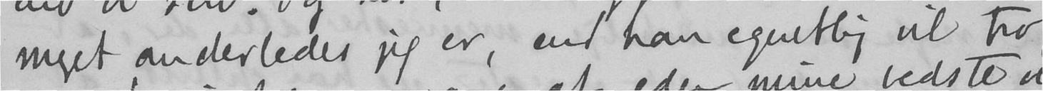meget anderledes jeg er, end han egentlig vil tro,
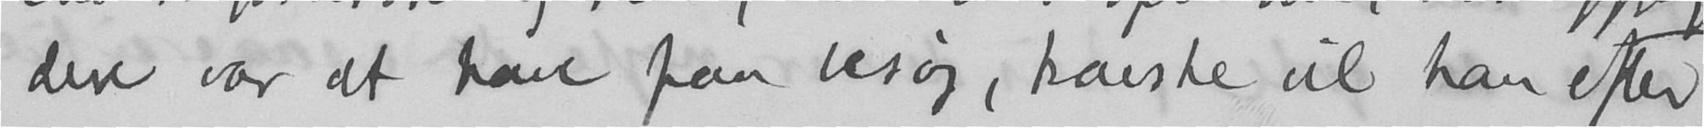dere var at have paa besøg, kanske vil han efter
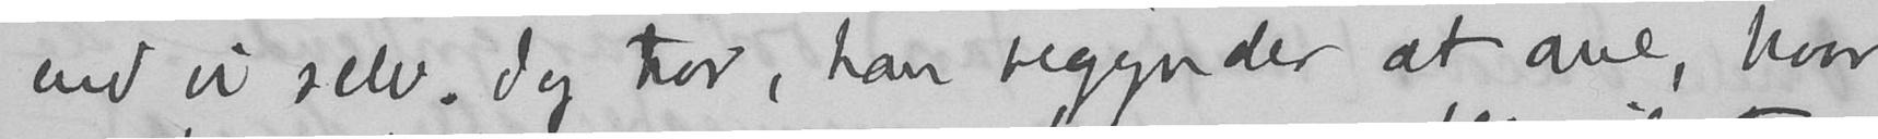end vi selv. Jeg tror, han begynder at ane, hvor
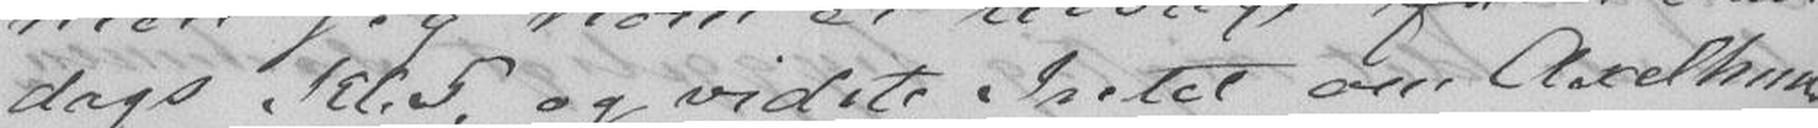dags Kl.5, og vidste Intet om Axelhuus
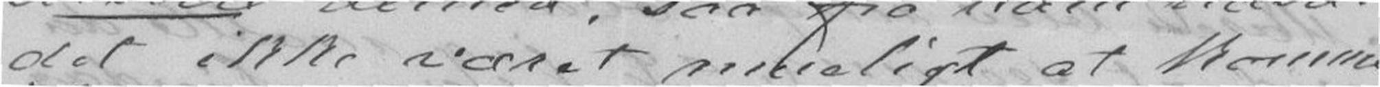det ikke været mueligt at komme
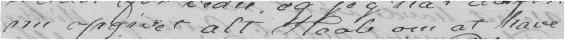nu opgivet alt Haab om at have
